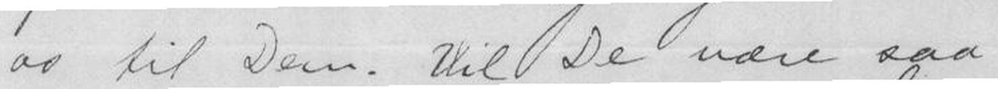os til Dem. Vil De være saa
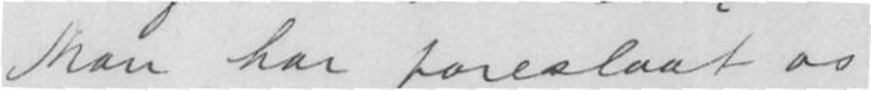Man har foreslaaet os
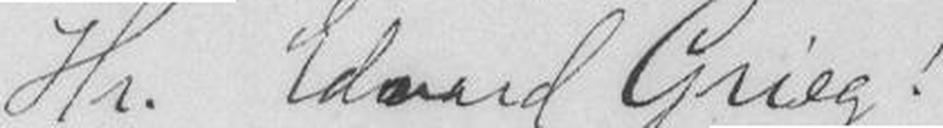Hr. Edvard Grieg!
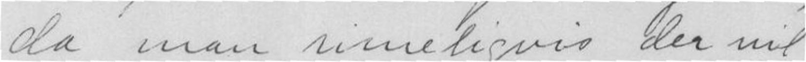da man rimeligvis der vil
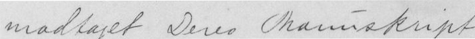modtaget Deres Manuskript;
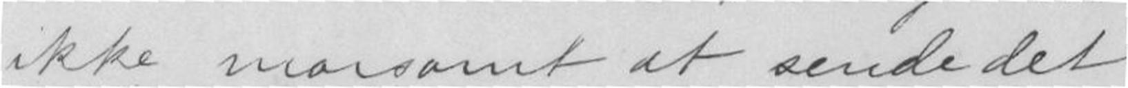ikke morsomt at sende det
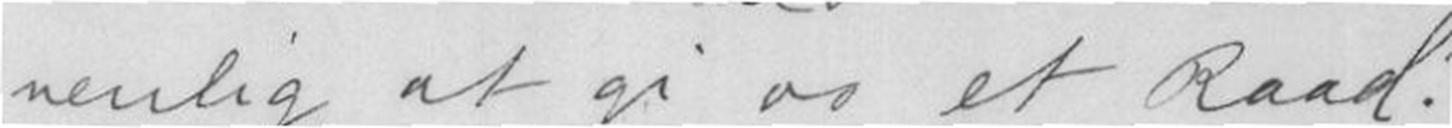venlig at gi os et Raad?
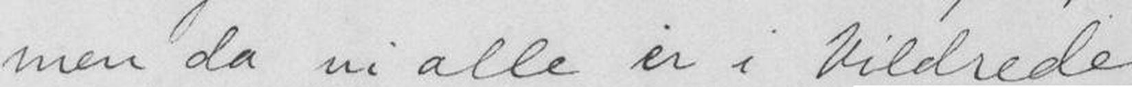men da vi alle er i Vilrede
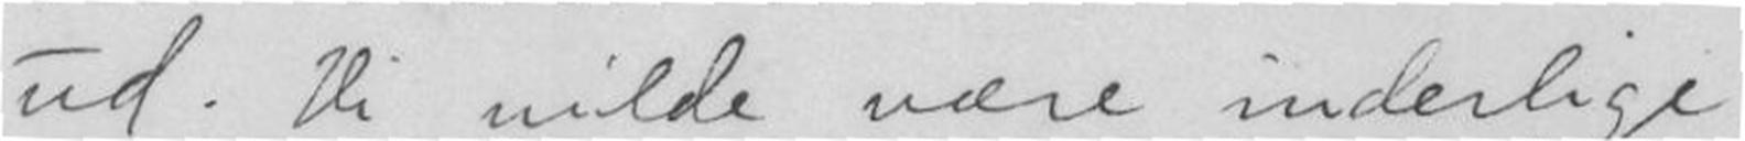ud. Vi vilde være inderlige
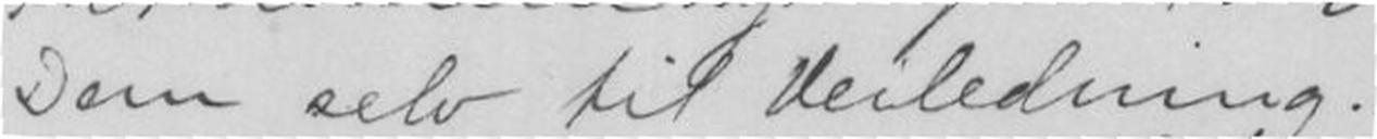Dem selv til Veiledning.
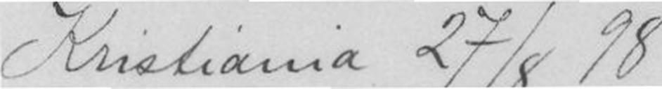Kristiania 27/8 98
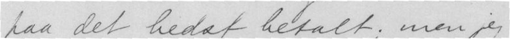faa det bedst betalt; men jeg
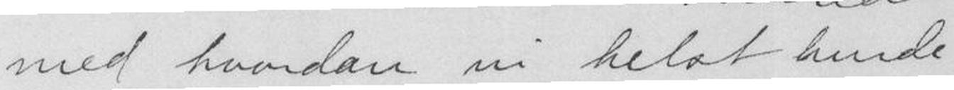med hvordan vi helst burde
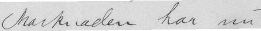Marknaden har nu
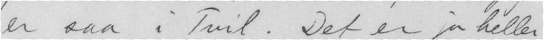er saa i Tvil. Det er jo heller
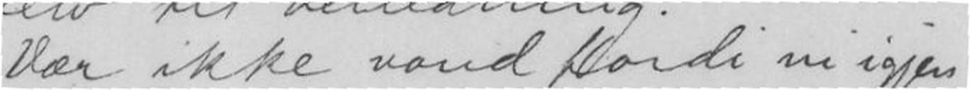Vær ikke vond fordi vi igjen
